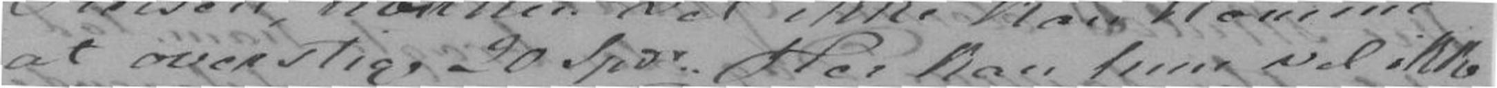at overstige vel 20 Spdo. Her kan hun vel ikke
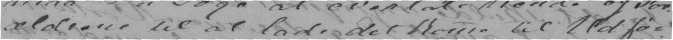ældrene til at lade det ko'me til udfø-
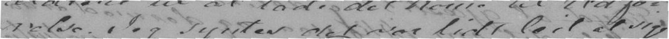relse. Jeg syntes det var lidt leit at sige
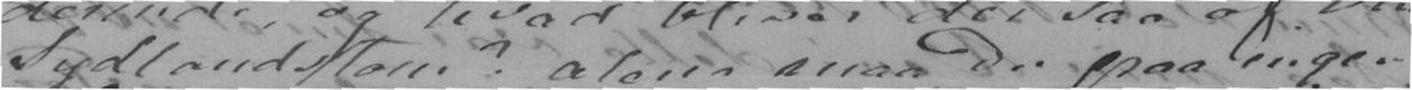Sydlandstour? alene maa Du paa ingen
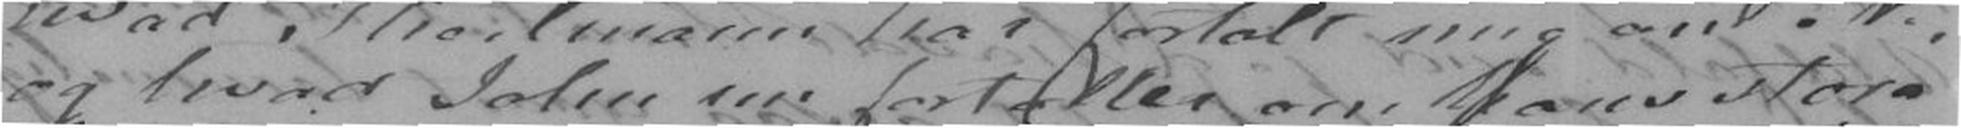og hvad John nu fortæller om hans store
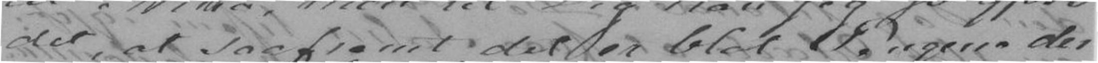det, at saafremt det er blot Pengene der
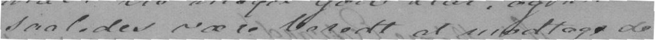saledes være beredt at modtage de
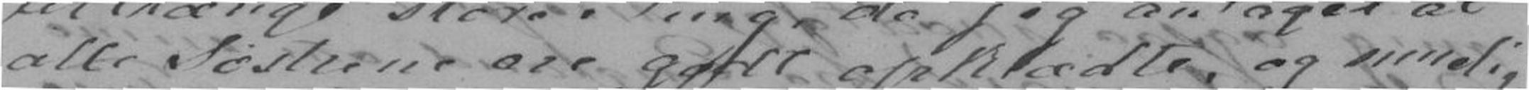alle søsterne ere godt opklædte, og muelig
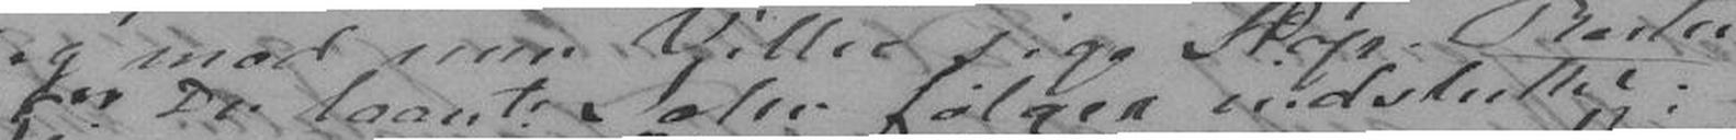25.. Du laante John følger indskutte.
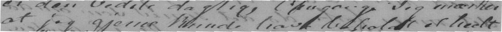at jeg gjerne kunde have beholdt et helt
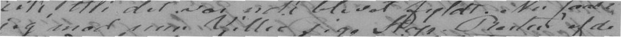jeg mod min Villie sige Stop. Resten af de
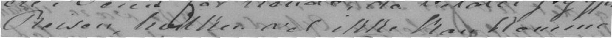Reisen, hvilken vel ikke kan komme
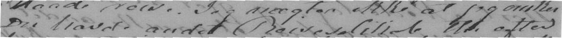Du havde andet Reiseselskab, thi efter
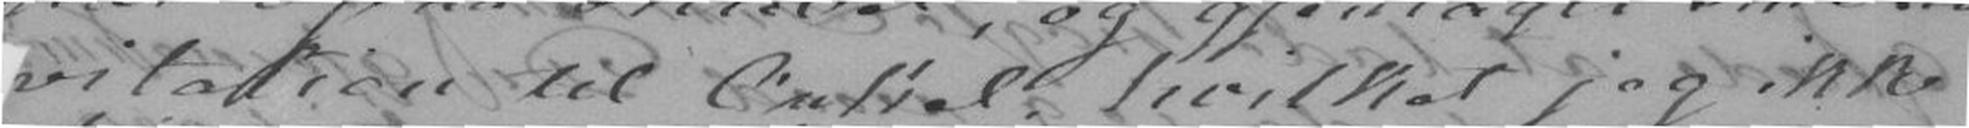vitation til Onkel, hvilket jeg ikke
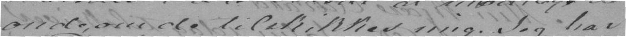onde. om det tilskikkes mig. Jeg har
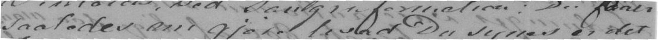saaledes nu gjøre hvad Du synes er det
